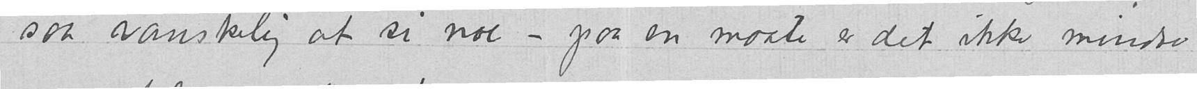saa vanskelig at si noe – paa en maate er det ikke mindre
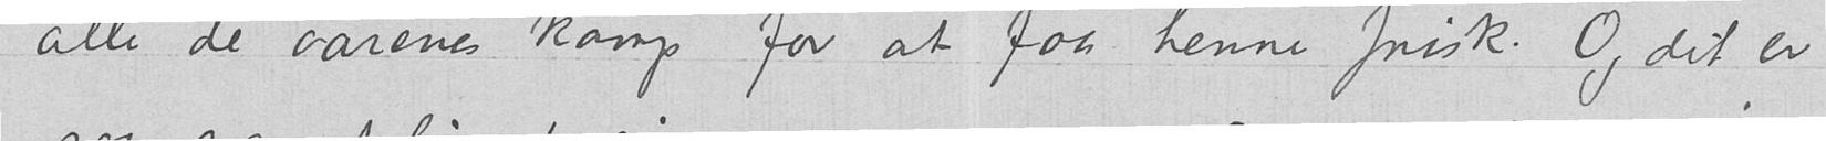alle de aarenes kamp for at faa henne frisk. Og det er
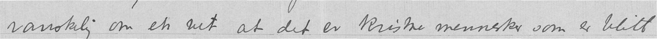vanskelig om en vet at det er kristne mennesker som er blitt
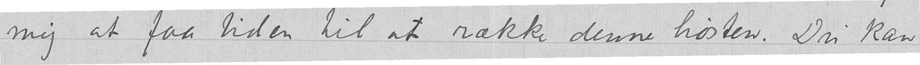mig at faa tiden til at række denne høsten. Du kan
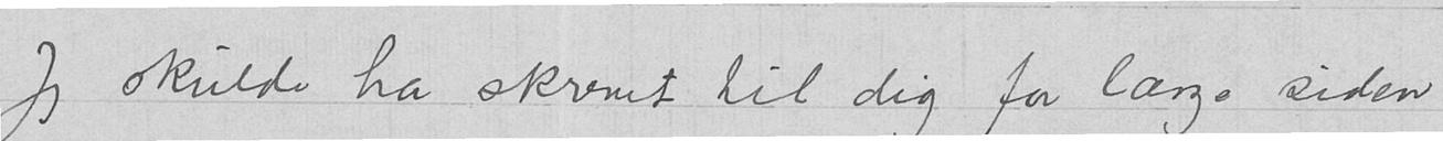Jeg skulde ha skrevet til dig for længe siden
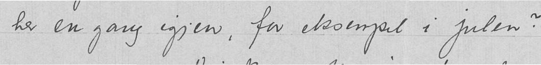her en gang igjen, for eksempel i julen?
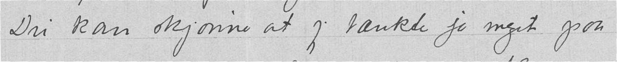Du kan skjønne at jeg tænkte jo meget paa
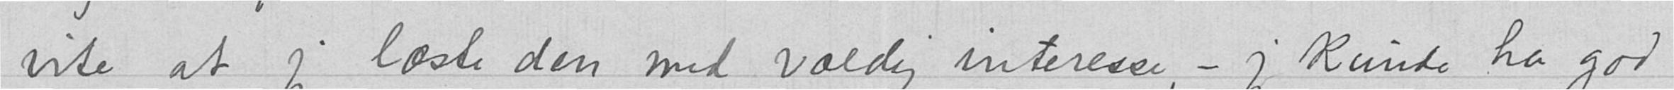vite at jeg læste den med vældig interesse, – jeg kunde ha god
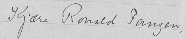Kjære Ronald Fangen,
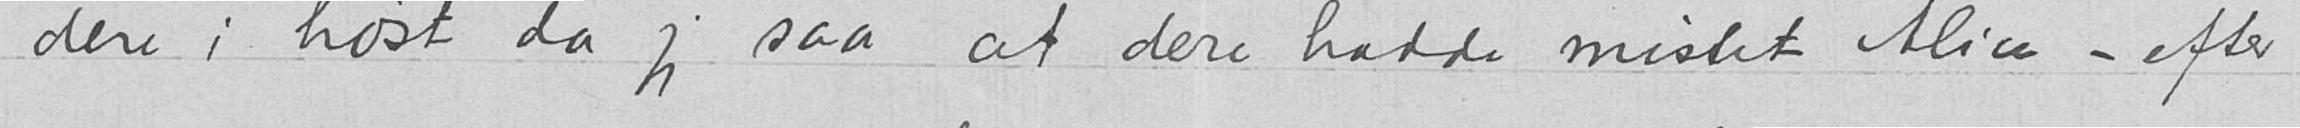dere i høst da jeg saa at dere hadde mistet Alice – efter
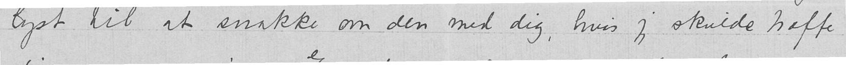lyst til at snakke om den med dig, hvis jeg skulde træffe
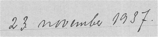23 november 1937.
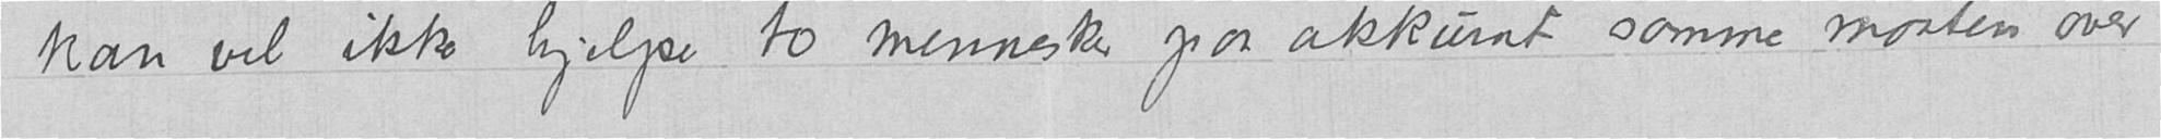kan vel ikke hjelpe to mennesker paa akkurat samme maaten over
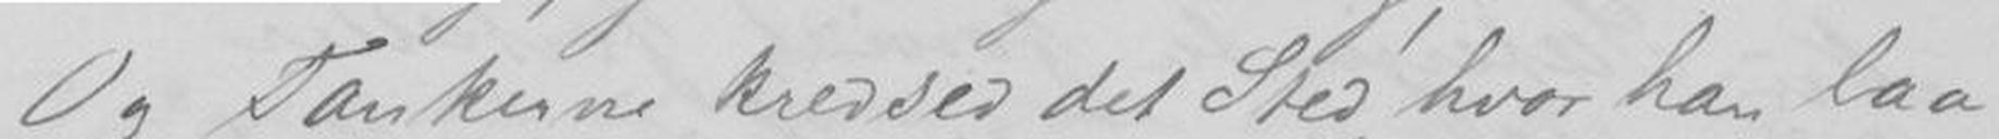Og Tankerne kredsed det Sted, hvor han laa
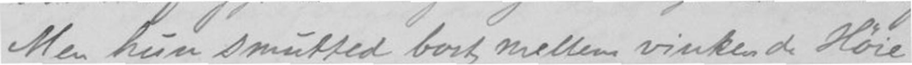Men hun smutted bort mellem vinkende Høie,
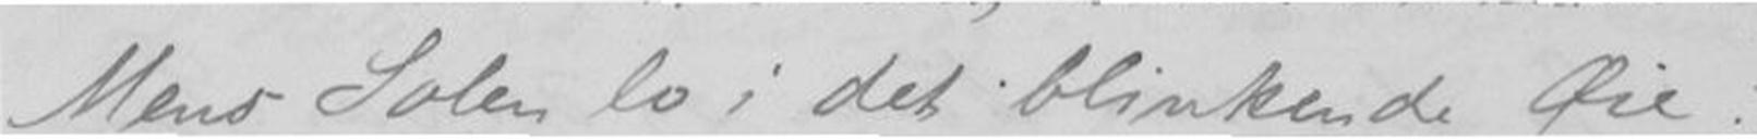Mens Solen lo i det blinkende Øie!
